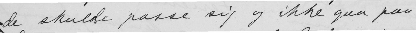de skulde passe sig og ikke gaa paa
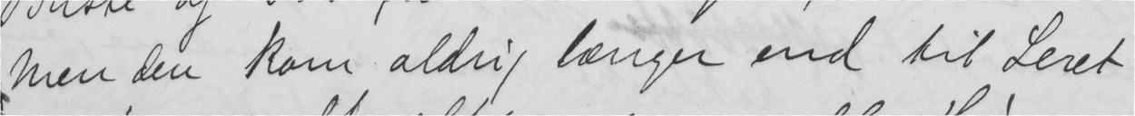Men den kom aldrig længer end til Leret
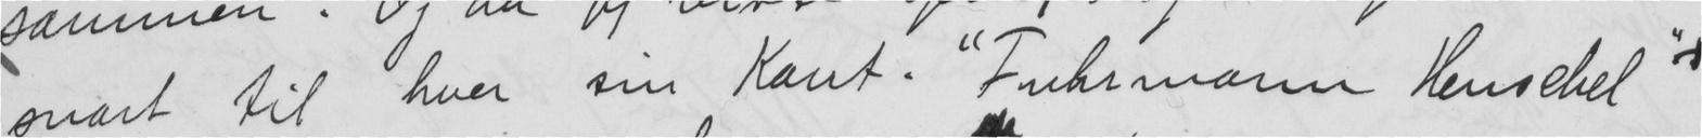snart til hver sin Kant. "Fuhrmann Henschel"
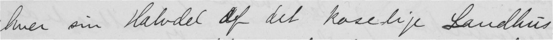hver sin Halvdel af det koselige Landhus
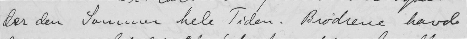der den Sommer hele Tiden. Brødrene havde
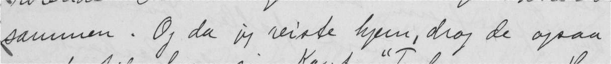sammen. Og da jeg reiste hjem, drog de ogsaa
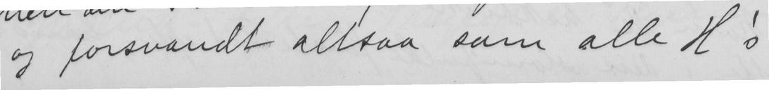og forsvandt altsaa som alle H!
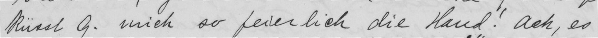kusst G. mich so feierlich die Hand! Ach, es
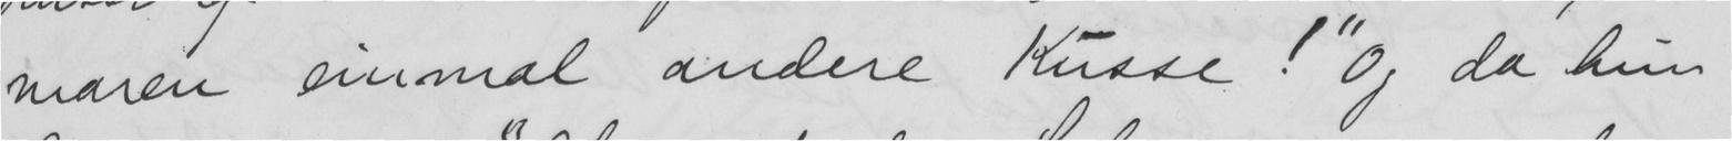maren einmal andere Kusse!" Og da hun
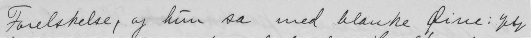Forelskelse, og hun sa med blanke Øine: Jebg
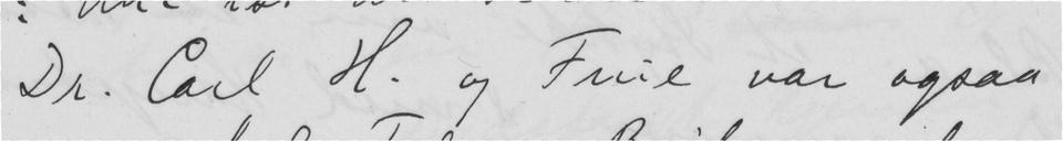Dr. Carl H. og Frue var ogsaa
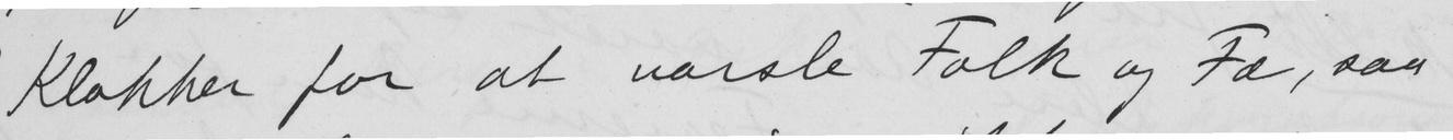Klokker for at varsle Folk og Fæ, saa
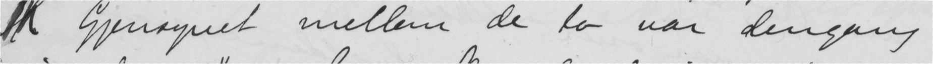Gjensynet mellem de to var dengang
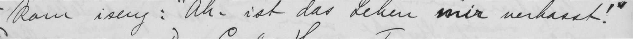kom iseng: "Ah- ist das Leben mir verhasst!"
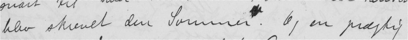blev skrevet den Sommer. Og en prægtig
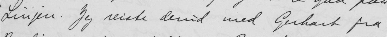Linjen. Jeg reiste derud med Gerhart fra
